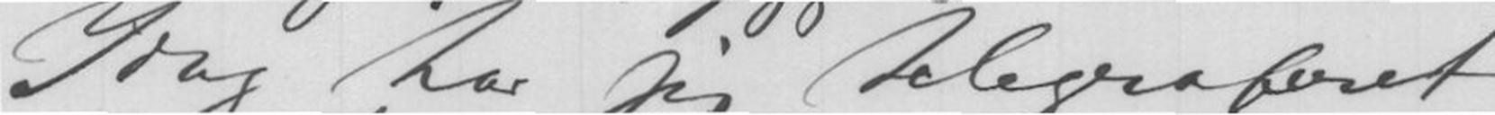Idag har jeg telegraferet
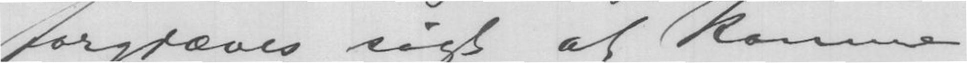forgjæves søgt at komme
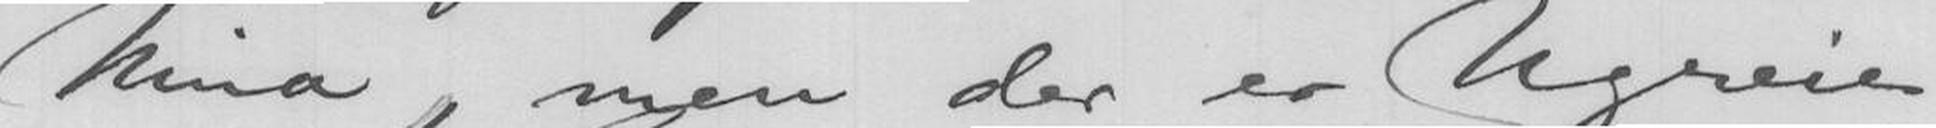Nina, men der er Ugreie
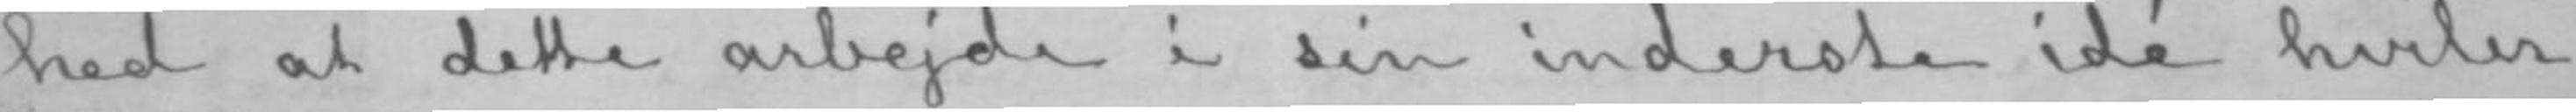hed at dette arbejde i sin inderste idé hviler
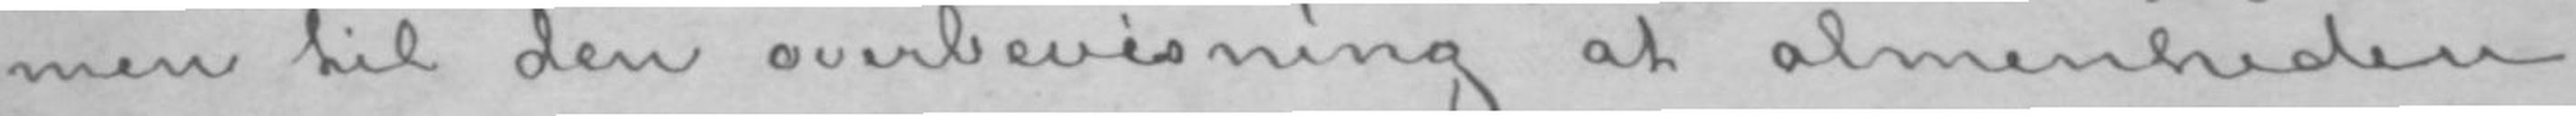men til den overbevisning at almenheden
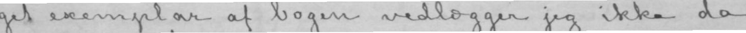get exemplar af bogen vedlægger jeg ikke da
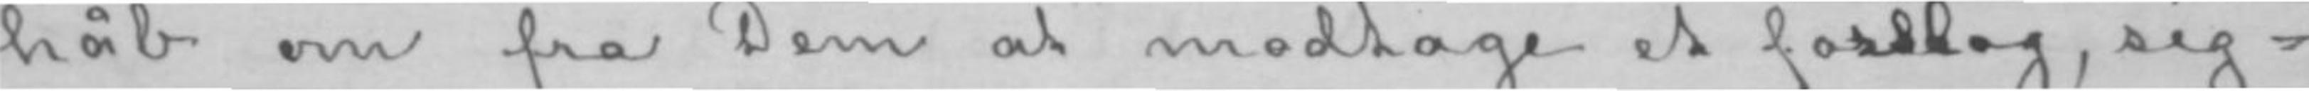håb om fra Dem at modtage et foslarslag, sig-
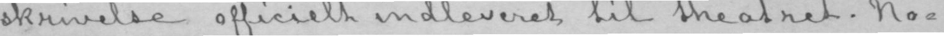skrivelse officielt indleveret til theatret. No-
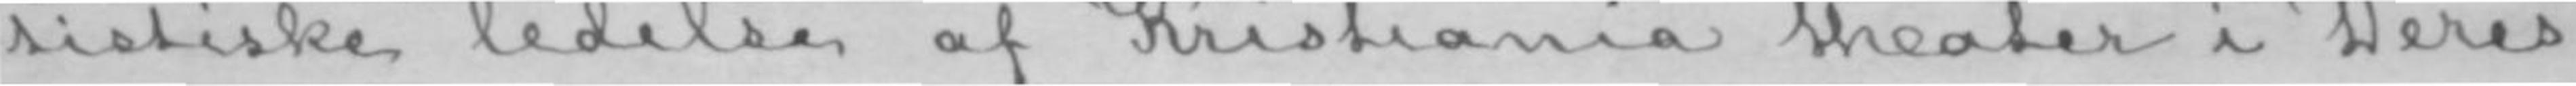tistiske ledelse af Kristiania theater i Deres
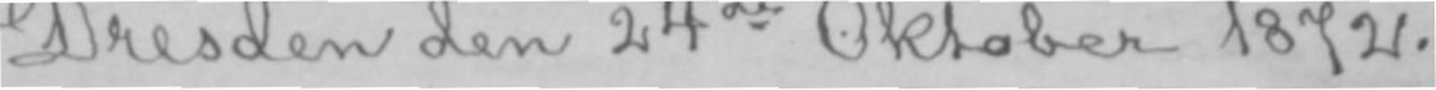Dresden den 24de Oktober 1872.-
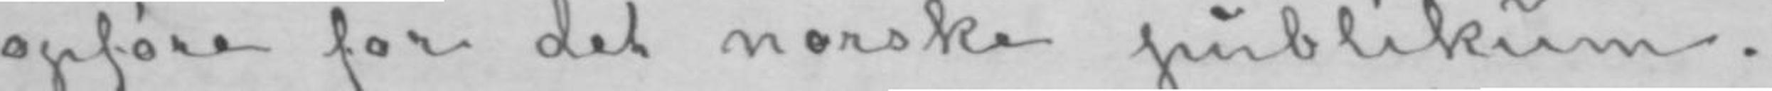opføre for det norske publikum.
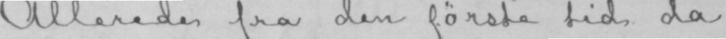Allerede fra den første tid da
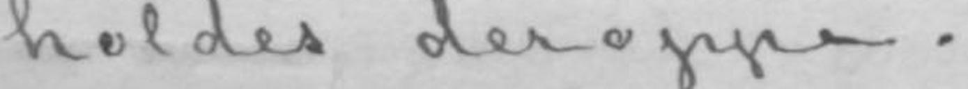holdes deroppe.
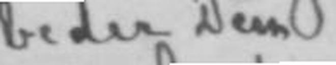beder Dem
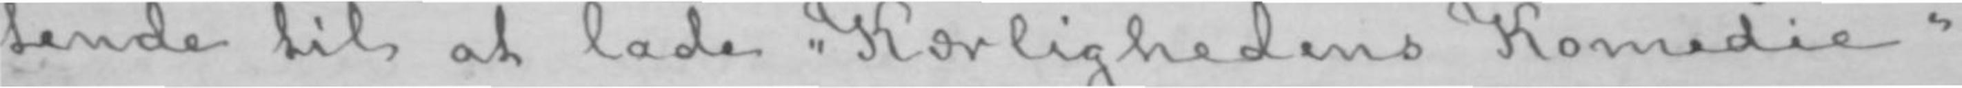tende til at lade «Kærlighedens Komedie»
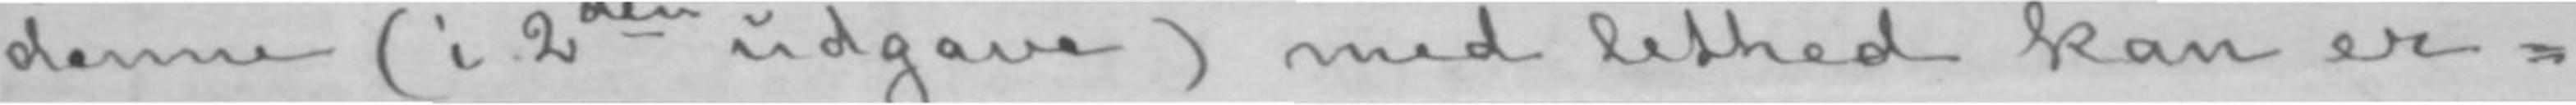denne (i 2den udgave) med lethed kan er-
